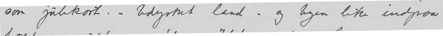som julekort. – Udyrket land – og byen like indpaa
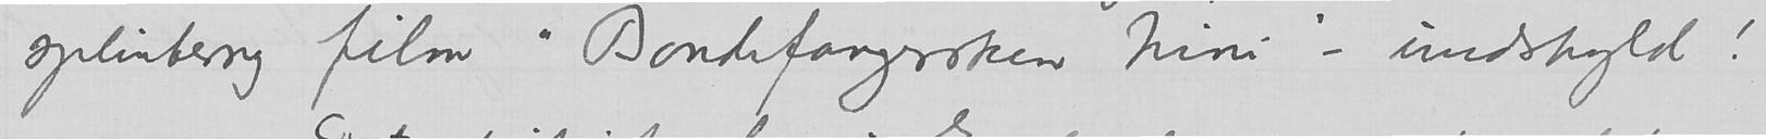splitterny film «Bondefangersken Nini» – undskyld!
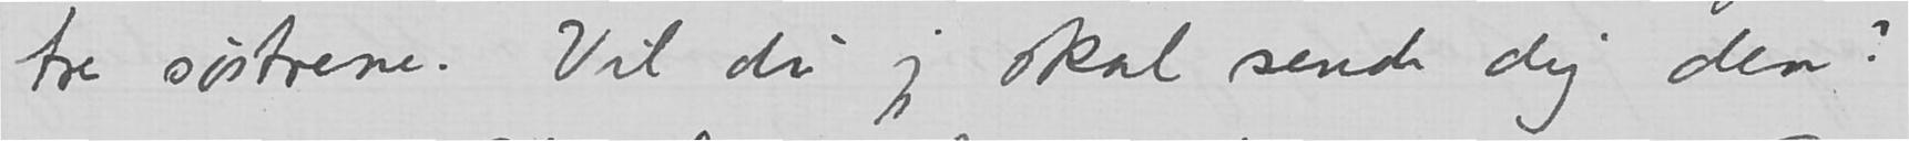tre søstrene. Vil du jeg skal sende dig den?
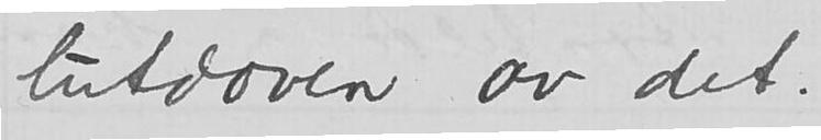lutdoven av det.
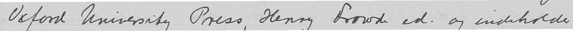Oxford University Press., Henry Frowde ed. og indeholder
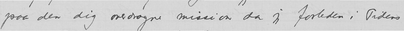paa den dig overdragne mission da jeg forleden i Tidens
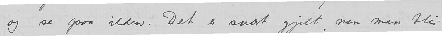og se paa ilden. Det er svært gjilt, men man blir
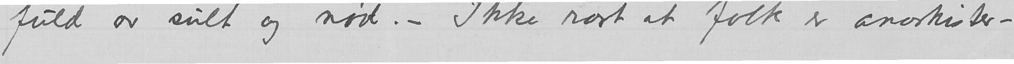fuld av sult og nød. – Ikke rart at folk er anarkister-
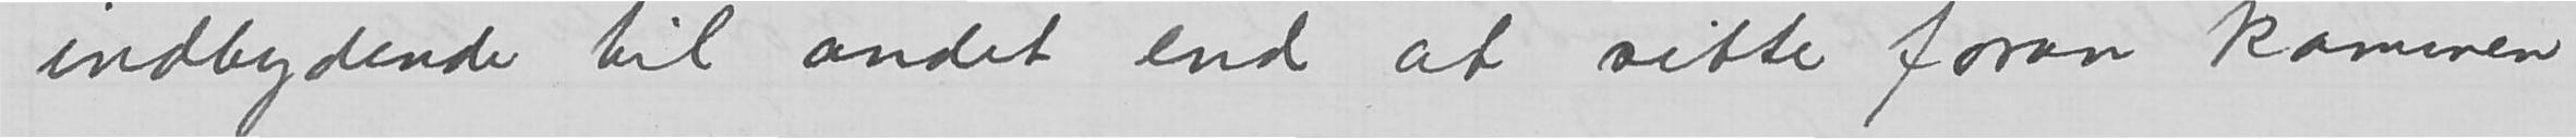indbydende til andet end at sitte foran kaminen
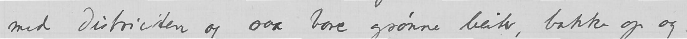med Disctrictern og saa bare grønne beiter, bakke op og
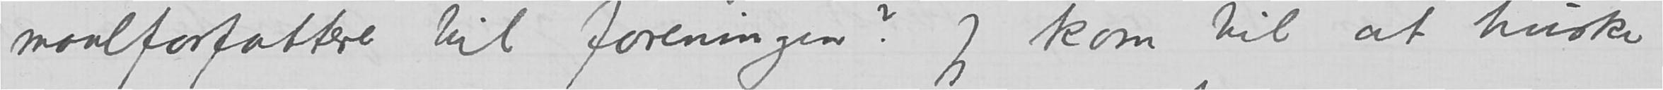maalforfattere til foreningen? Jeg kom til at huske
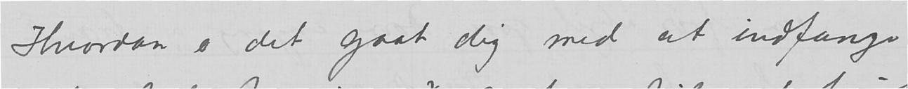Hvordan er det gaat dig med at indfange
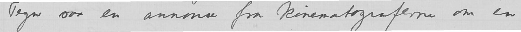Tegn saa en annonse fra kinematograferne om en
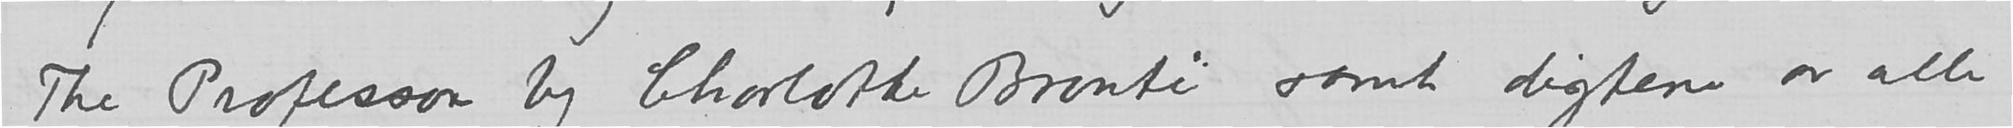The Professor by Charlotte Brontë samt digtene av alle
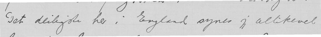Det deiligste her i England synes jeg allikevel
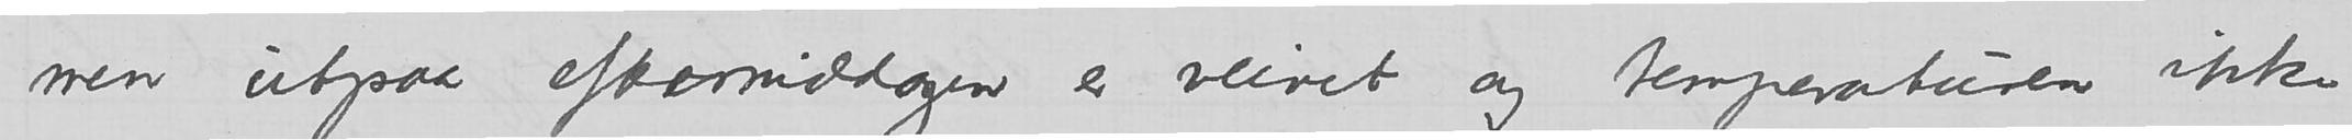men utpaa eftermiddagen er veiret og temperaturen ikke
